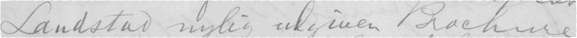Landstad nylig udgiver Brochure
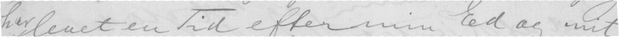har levet en Tid efter min Ed og mit
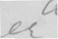er
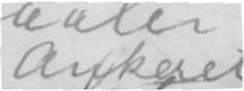Ankeret
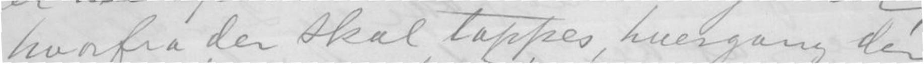hvorfra Der skal tappes, hvergang der
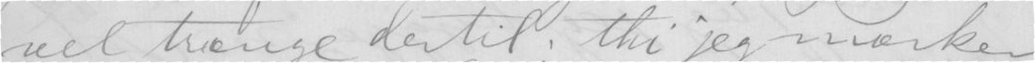vel trænge dertil; thi jeg mærker
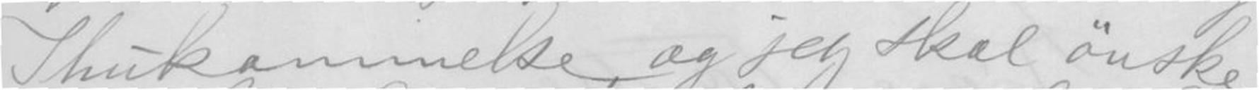Ihukommelse, og jeg skal ønske
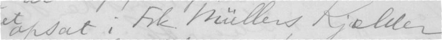oppsat i Frk Müllers Kjælder
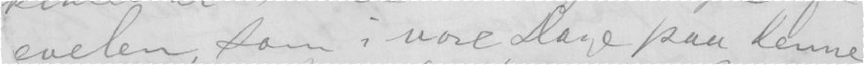evelen, som i vore Dage paa denne
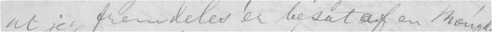at jeg fremdeles er besat af en Mængde
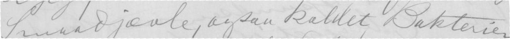Smaadjævle, ogsaa kaldet Bakterier
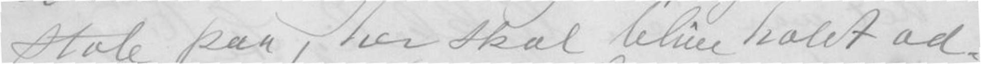stole paa, her skal blive holdt ad-
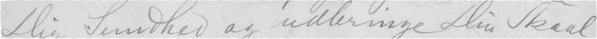Dig Sundhed og udbringe Din Skaal
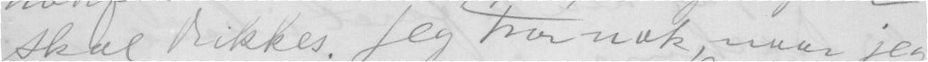skal drikkes. Jeg tror nok, naar jeg
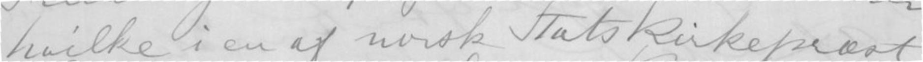hvilke i en af norsk Statskirkepræst
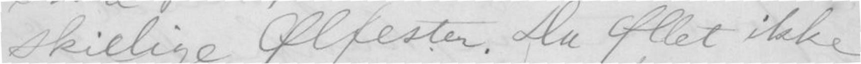skillige Ølfester. Da Øllet ikke
